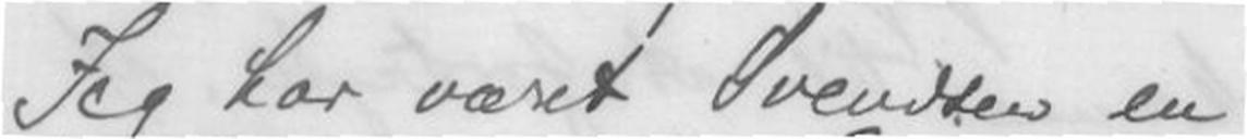Jeg har været Svendsen en
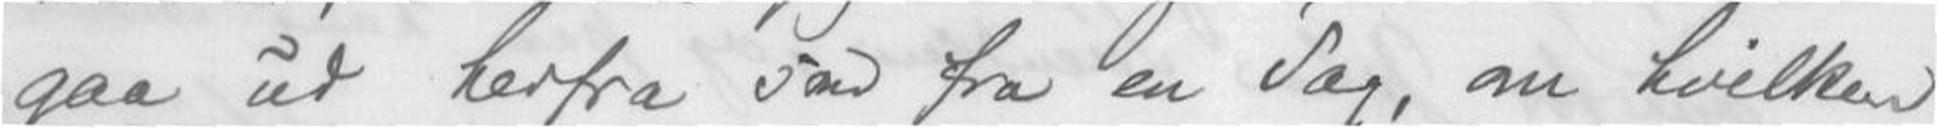gaa ud herfra fra en Sag, om hvilken
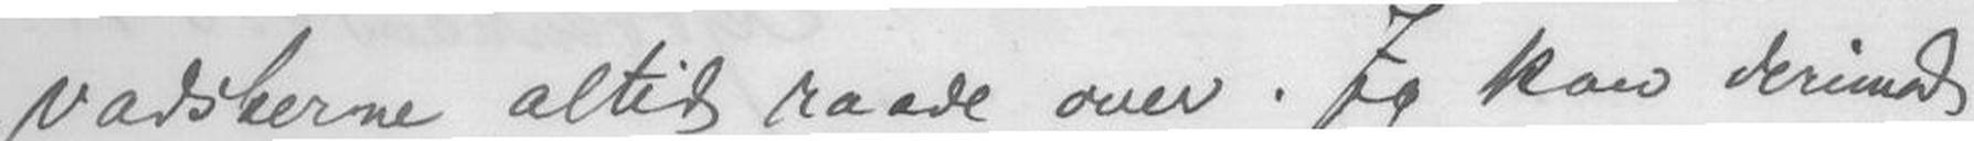vaskerne altid raade over. Jeg kan derinde
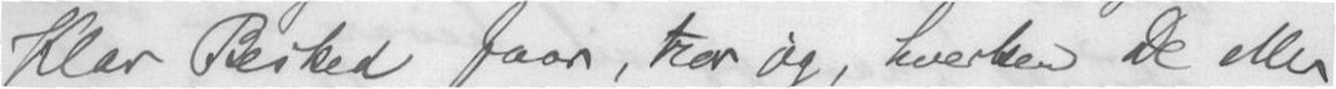Klar Besked faar , tror og, hverken De eller
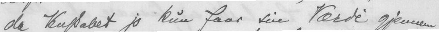da Venskabet jo kun faar sin Værdi gjennem
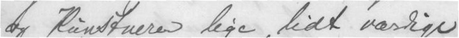og Kunstnere lige lidt værdige
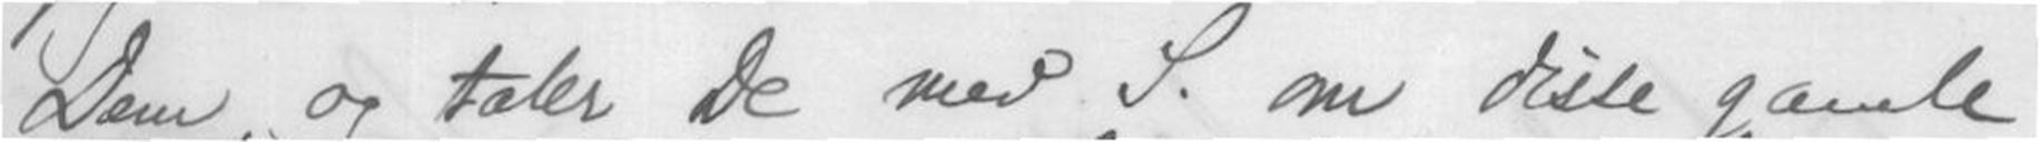Dem, og taler de med S. om gamle
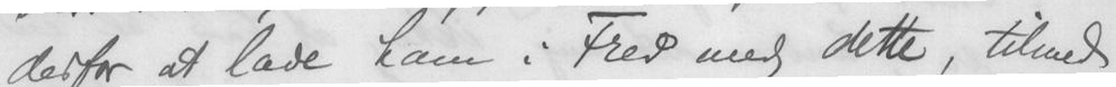derfor at lade ham i Fred med dette, tilmed
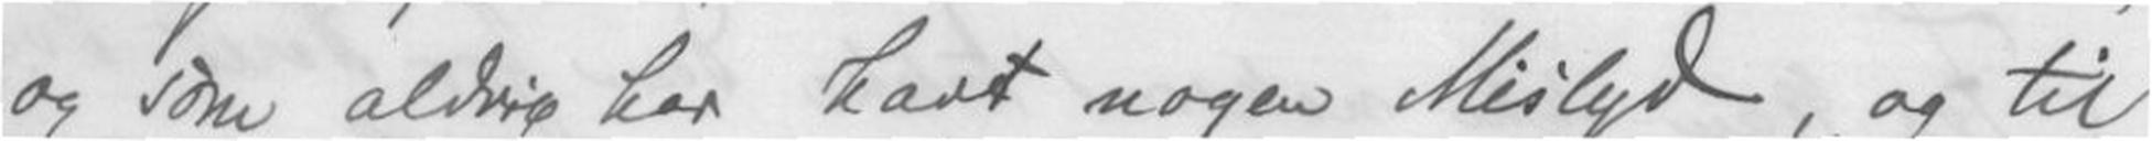og som aldrig har havt nogen Mislyd, og til
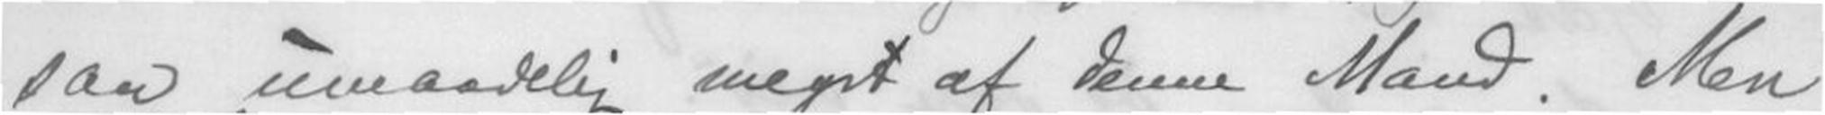saa umaadelig meget af denne Mand. Men
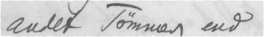andet Tømmer end
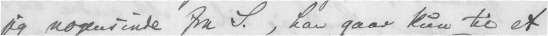jeg nogensinde fra S., han gaar kun til et
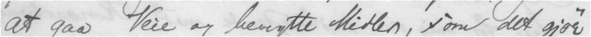at gaa Veie og benytte midler, som det gjøre
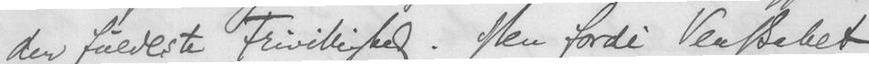den fuldeste Frivillighed. Men fordi Venskabet
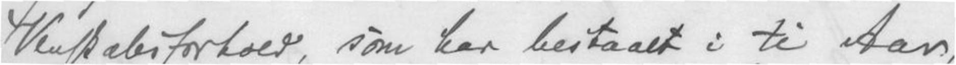Venskabsforhold, som har bestaaet i ti Aar,
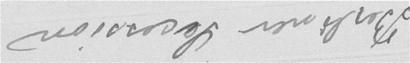Berliner Secession
